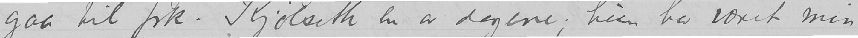gaa til frk. Kjølseth en av dagerne; hun har været min
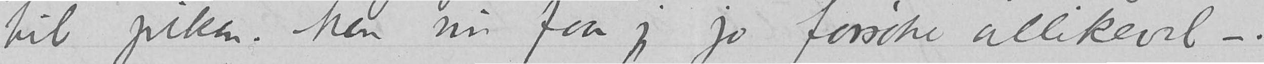til piken. Men nu faar jeg jo forsøke allikevel -.
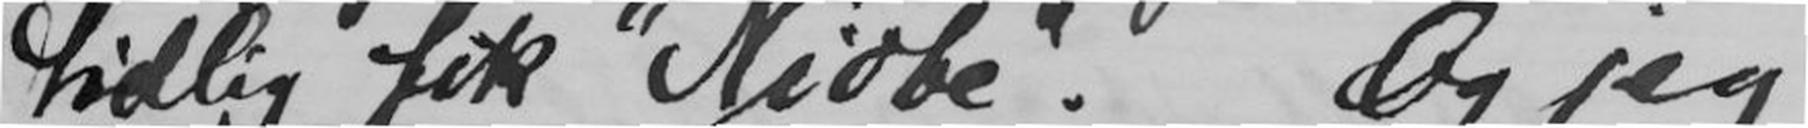tidlig fik "Niobe".Og jeg
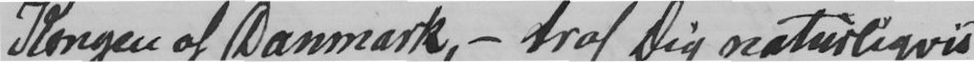Kongen af Danmark, - traf Dig naturligvis
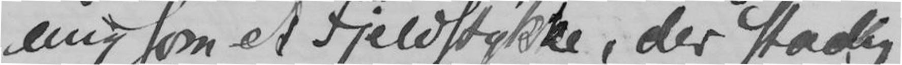mig som et Fjeldstykke, der stadig
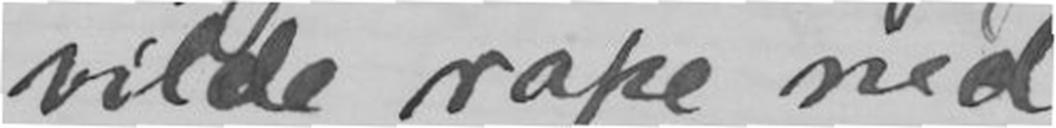vilde rase ned.
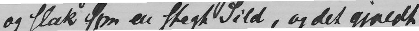og slak som en stegt Sild, og det gjaldt
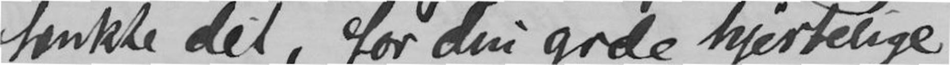tænkte det, for din gode hjertelige
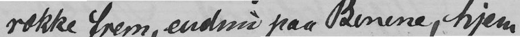rokke frem, endnu paa Benene, hjem
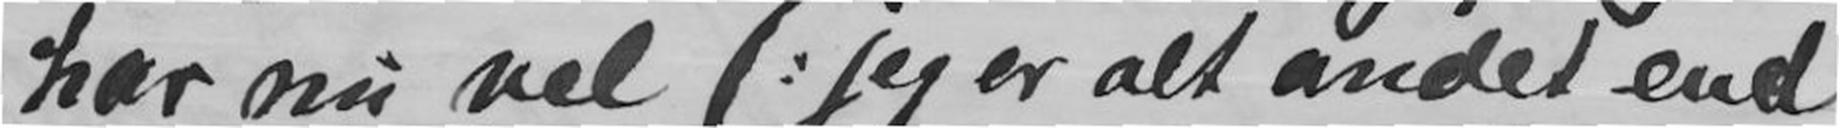har nu vel (jeg er alt andet end
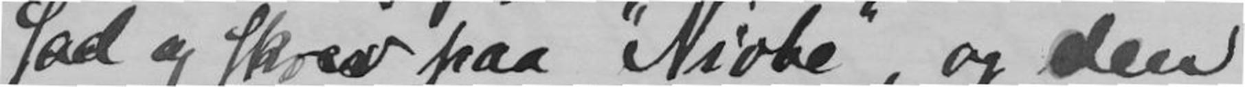sad og skrev paa "Niobe", og den
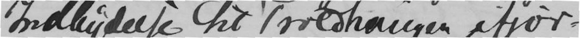Indbydelse til Troldhaugen ifjor-
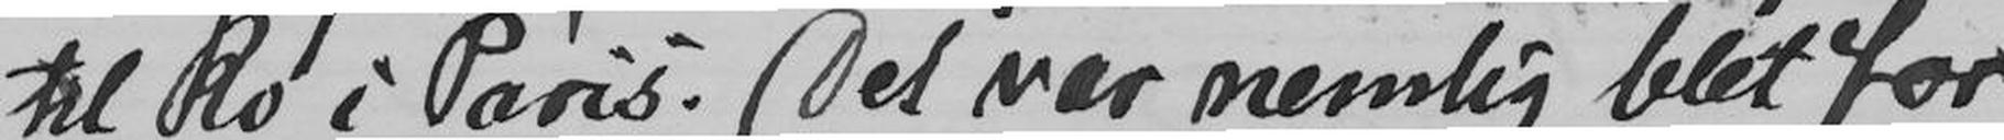til Ro i Paris. Det var nemlig blot for
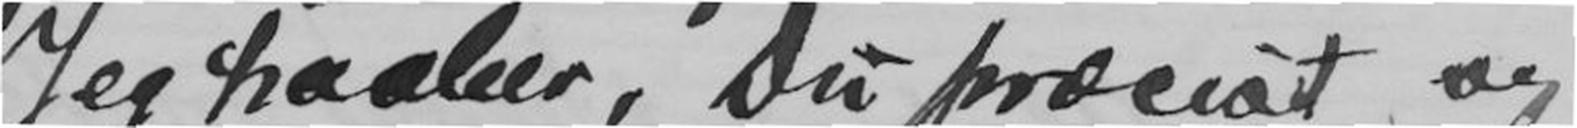Jeg haaber, Du præcist og
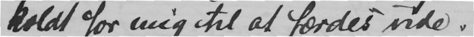koldt for mig til at færdes ude.
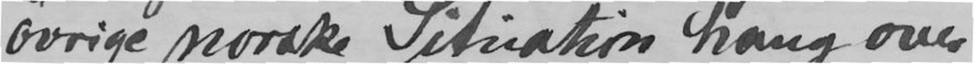øvrige norske Situation hang over
